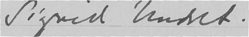Sigrid Undset
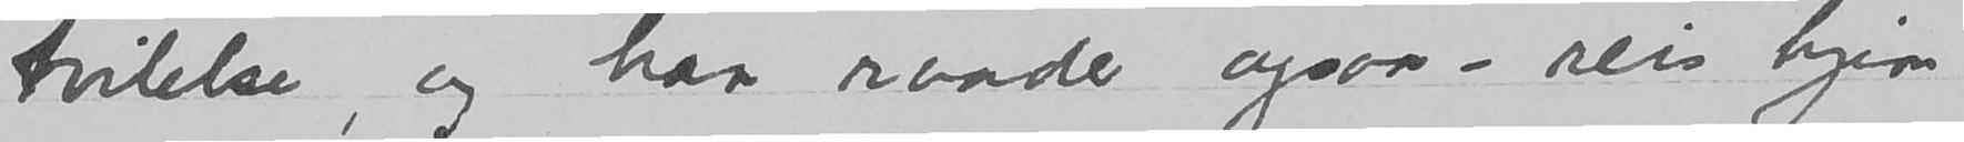tvilelse, og han raader ogsaa – reis hjem
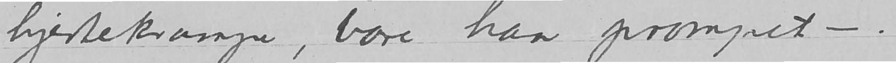hjertekrampe, bare han prompet –
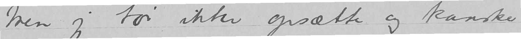Men jeg tør ikke opsætte og kanske
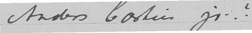Anders Castus jr.?
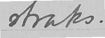straks.
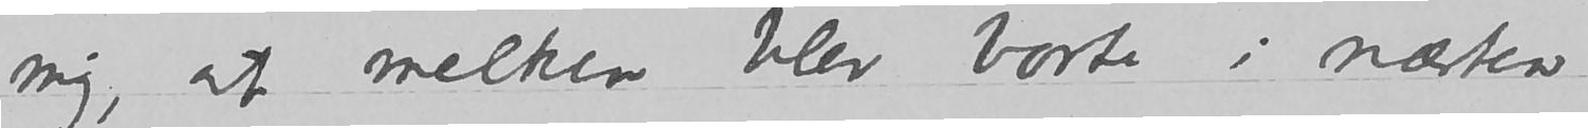mig, at melken blev borte i næsten
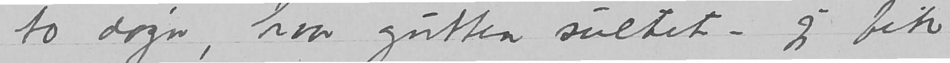to døgn, hvor gutten sultet – jeg fik
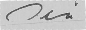Din
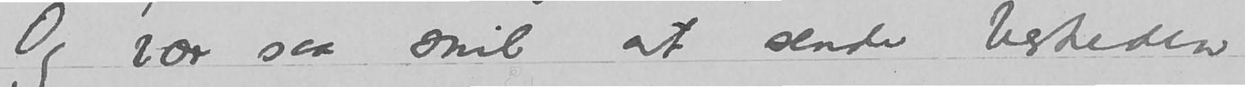Og vær saa snil at sende beskeden
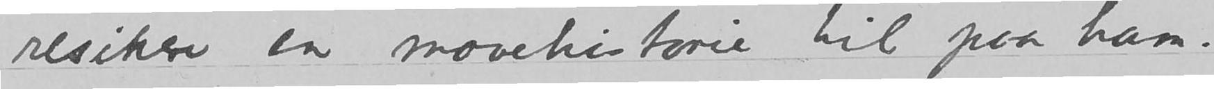resikere en mavehistorie til paa ham.
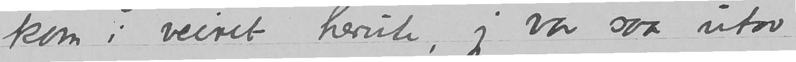kom i veiret herute, jeg var saa utav
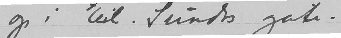op til Eil. Sundts gate.
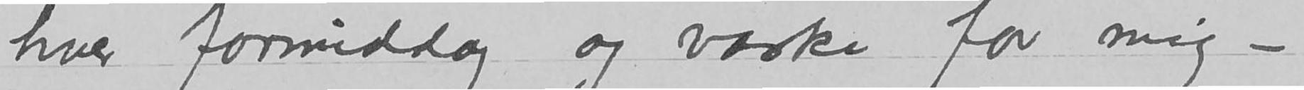hver formiddag og vaske for mig –
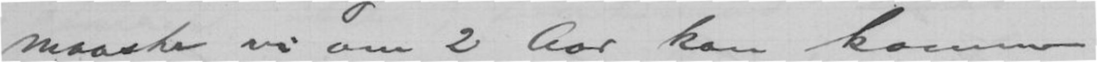maaske vi om 2 Aar kan komme
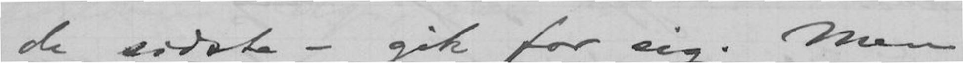- de sidste - gik for sig. Men
